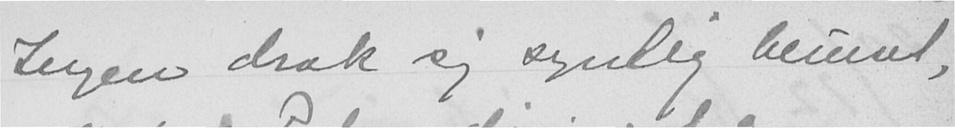Ingen drak sig synlig beruset,
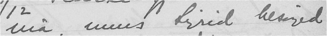nia, mens Sigrid besørged
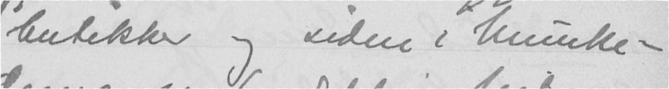butikker og siden i Munke-
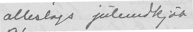alleslags juleindkjøb
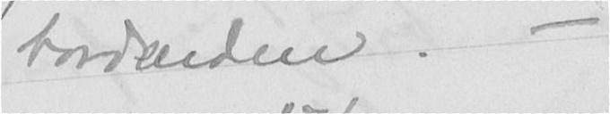bordenden. -
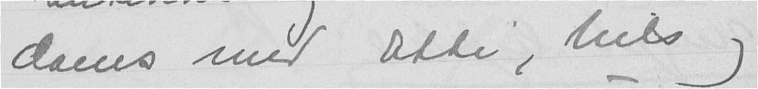dams med Etti, Nils og
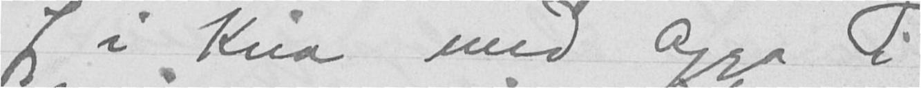Jeg i Kria med Agga i
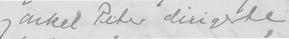og onkel Peter dirigerte
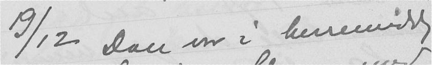19/12 Don var i herremiddag
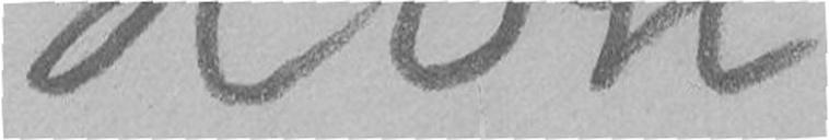Høn
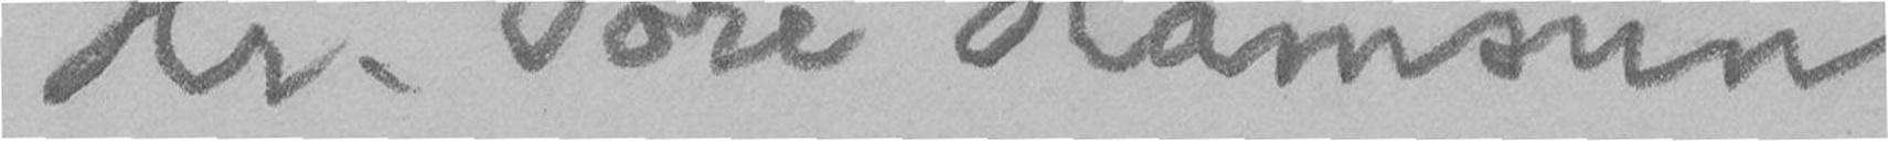Hr. Tore Hamsun
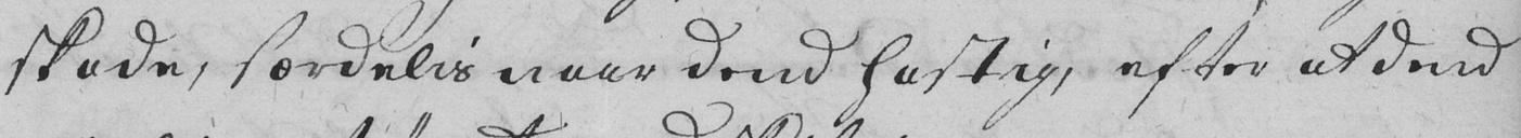skade, særdelis naar dend hastig, efter at dend
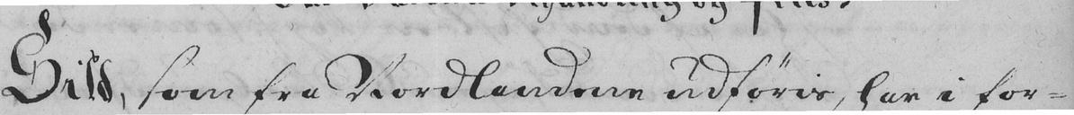Sild, som fra Nordlandene udføris, har i for-
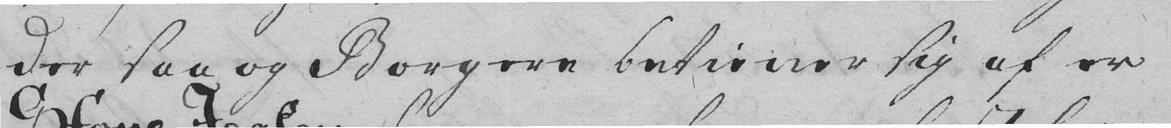der saa og Borgere betiener sig af er
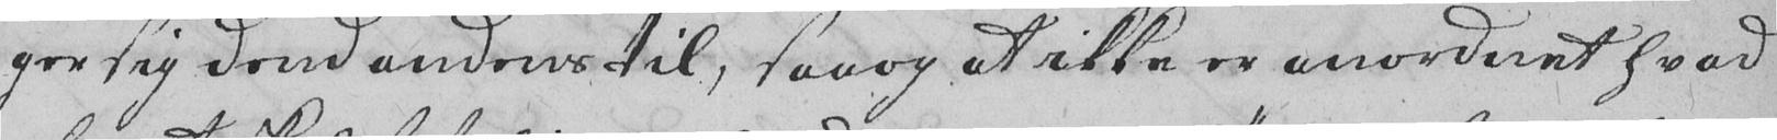ger sig dend andens til, saa og at ikke er anordnet hvad
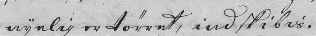nyelig er tørret, indskibis.
